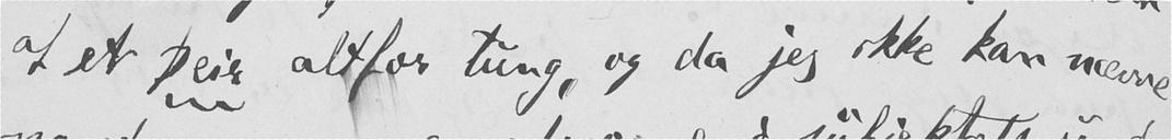af et Þeir altfor tung, og da jeg ikke kan nævne
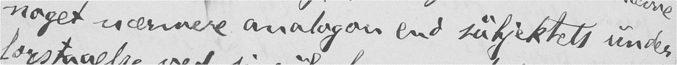noget nærmere analogon end subjektets under-
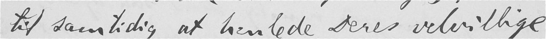til samtidig at henlede Deres velvillige
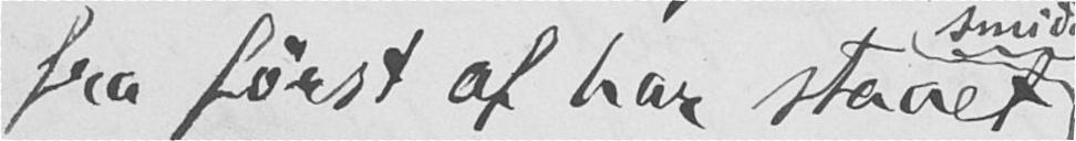fra først af har staaet
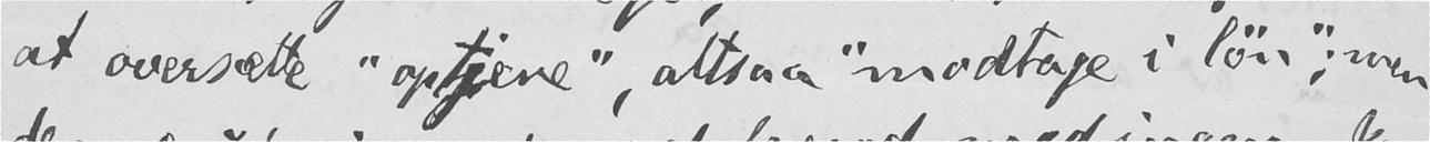at oversætte "optjene", altsaa "modtage i løn"; men
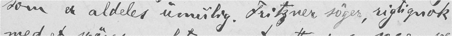som er aldeles umulig. Fritzner søger, rigtignok
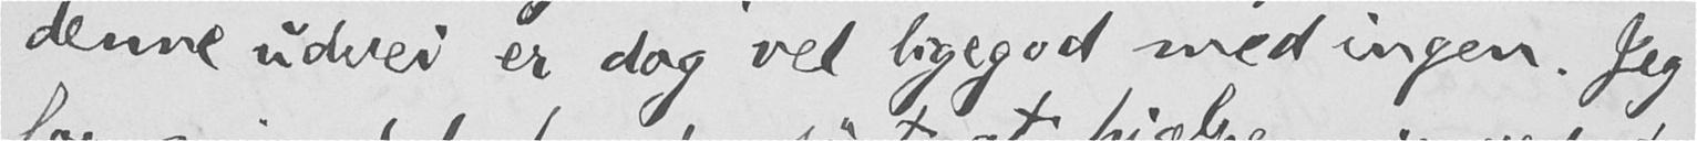denne udvei er dog vel ligegod med ingen. Jeg
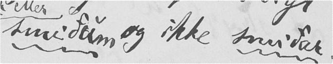smiðum, og ikke smiðar;
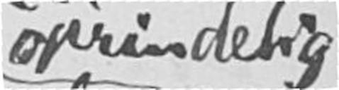oprindelig
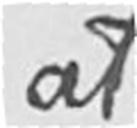at
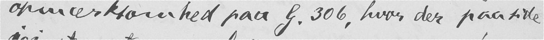opmærksomhed paa G. 306, hvor der paa side
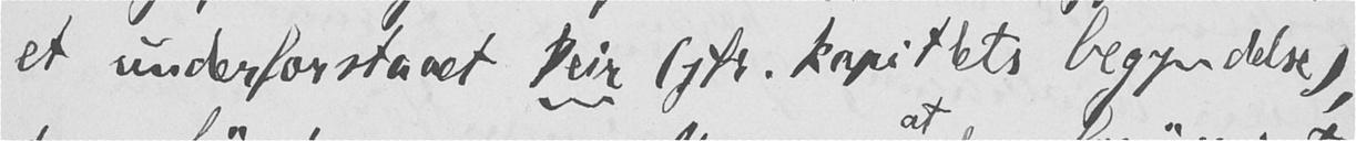et underforstaaet Þeir (jfr. kapitlets begyndelse)
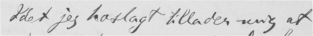Idet jeg hoslagt tillader mig at
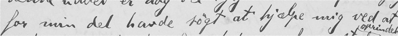for min del havde søgt at hjælpe mig ved at
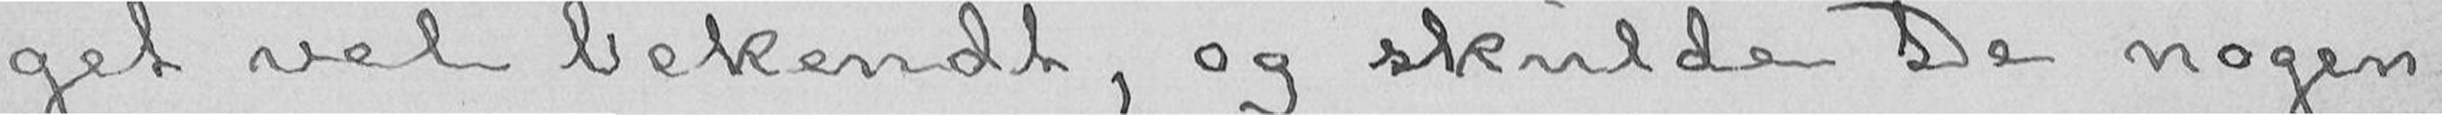get vel bekendt, og skulde De nogen-
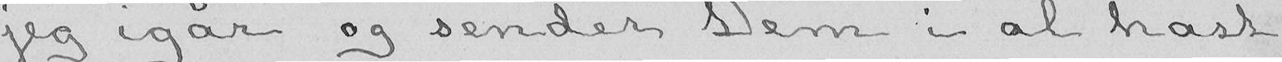jeg igår og sender Dem i al hast
